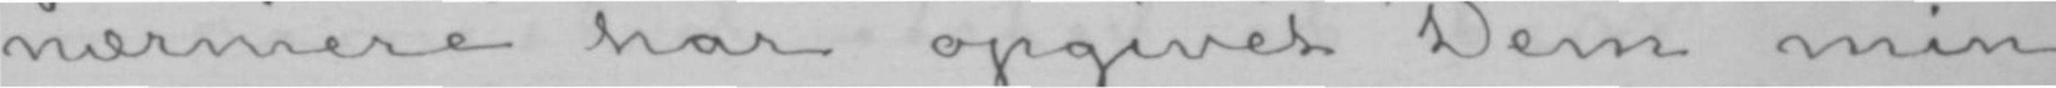nærmere har opgivet Dem min
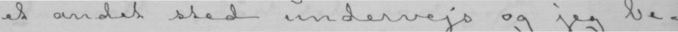et andet sted undervejs og jeg be-
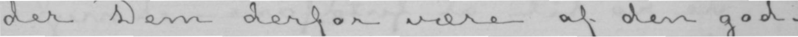der Dem derfor være af den god-
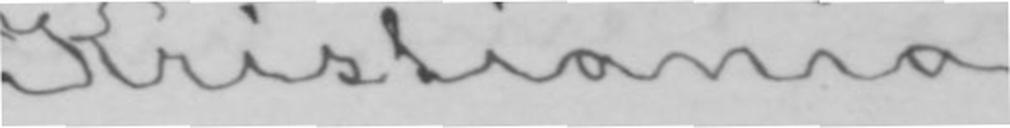Kristiania.
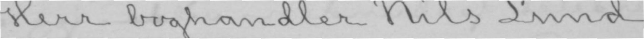Herr boghandler Nils Lund.
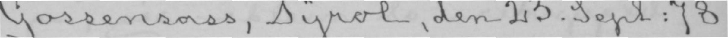Gossensass, Tyrol, den 23. Sept: 78.
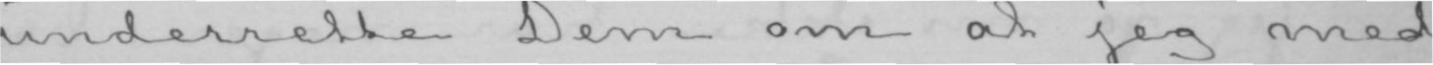underrette Dem om at jeg med
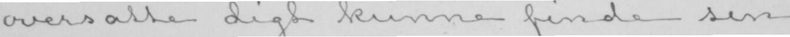oversatte digt kunne finde sin
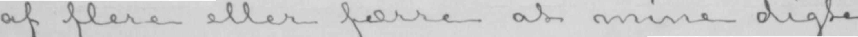af flere eller færre at mine digte,
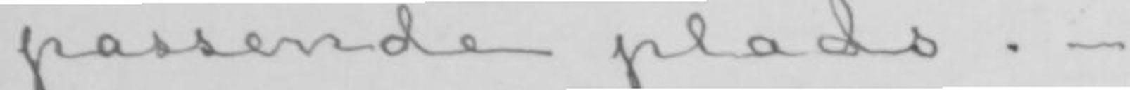passende plads.-
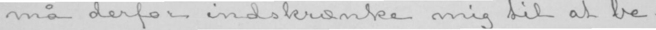må derfor indskrænke mig til at be-
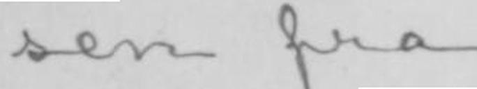sen fra
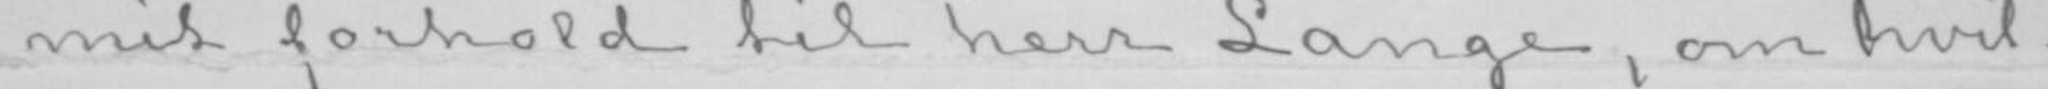mit forhold til herr Lange, om hvil-
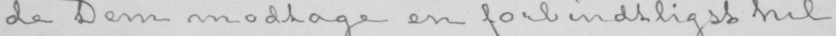de Dem modtage en forbindtligst hil-
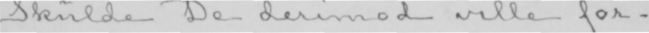Skulde De derimod ville for-
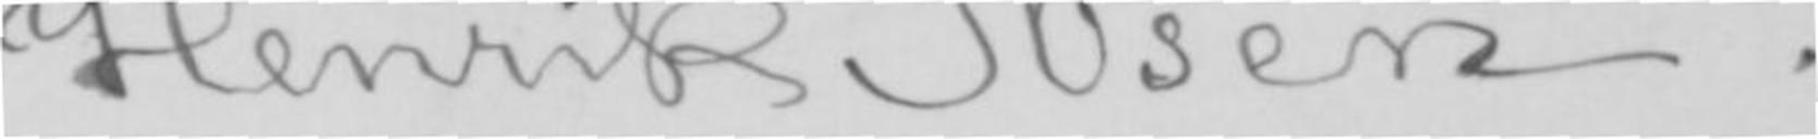Henrik Ibsen.
